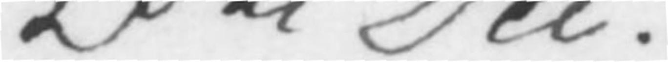2den Dec.
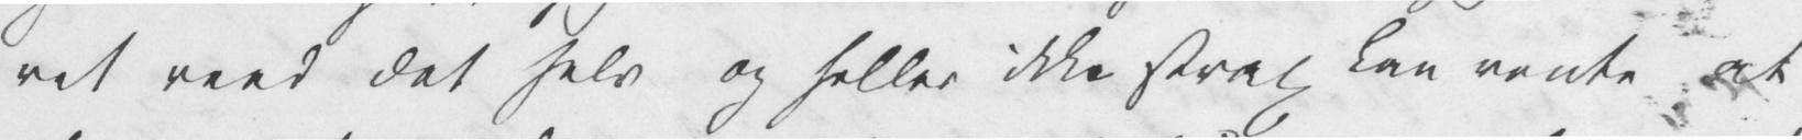ret veed det selv og heller ikke strax kan vente at
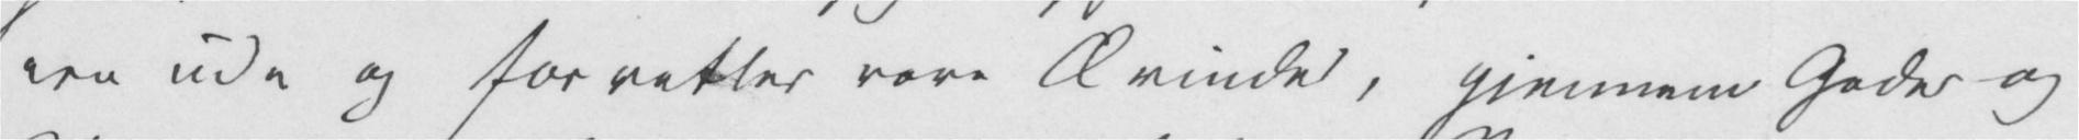ere ude og forretter vore Ærinder, gjennem Gader og
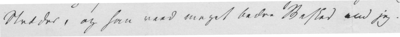Stræder, og han veed meget bedre Besked end jeg.
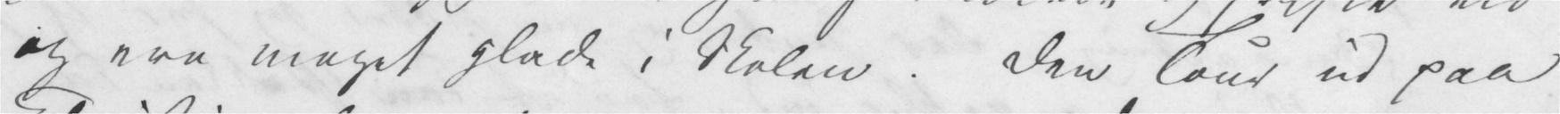og ere meget glade i Skolen. Den Tour ud paa
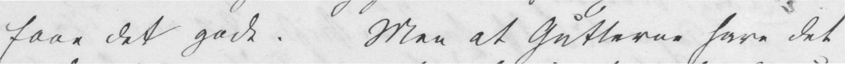faae det godt. Men at Gutterne have det
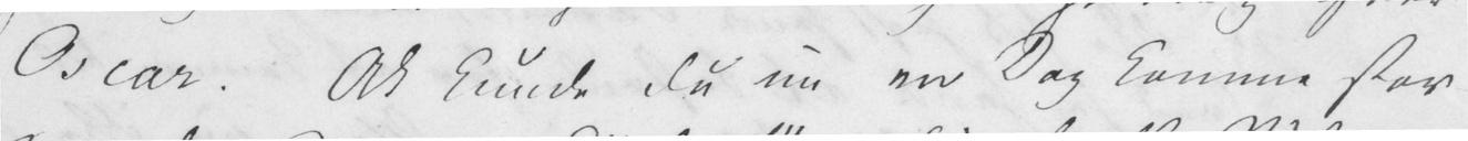Oscar. Ak kunde Du nu en Dag komme stor-
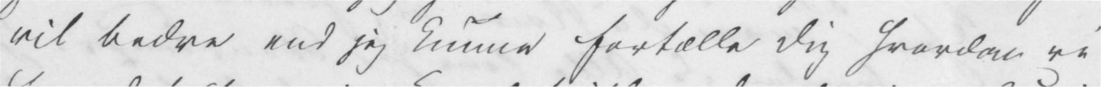vil bedre end jeg kunne fortælle Dig hvordan vi
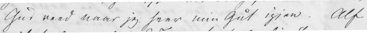Gud veed naar jeg seer min Gut igjen. Alf
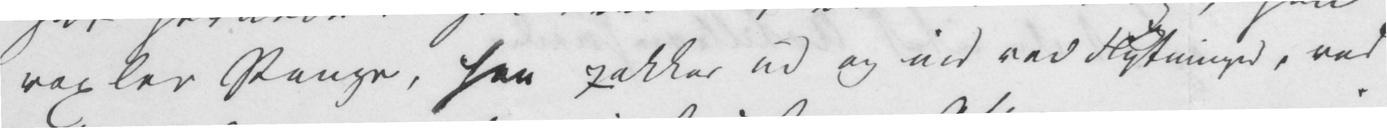vexler Penge, han pakker ud og ind ved Flytninger, ved
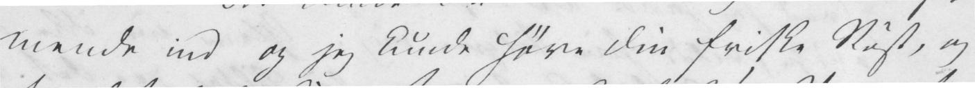mende ind og jeg kunde høre Din friske Røst, og
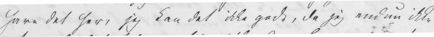have det her, jeg kan det ikke godt, da jeg endnu ikke
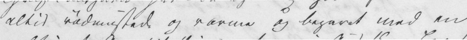altid rødmussede og varme og begavet med en
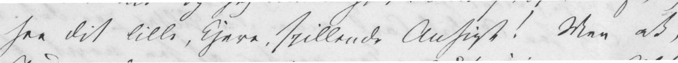see Dit lille, kjere, spillende Ansigt! Men ak,
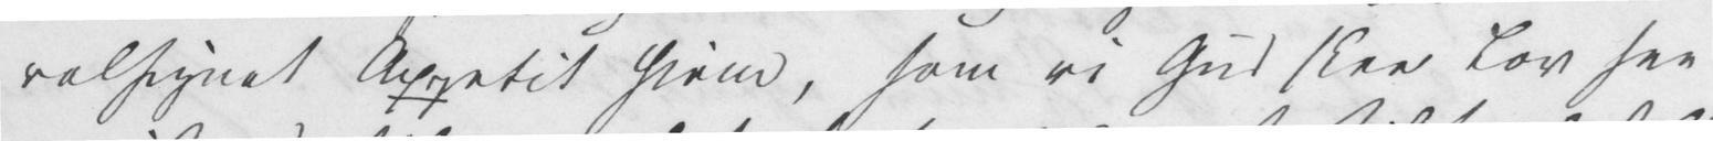velsignet Appetit hjem, som vi Gud skee Lov see
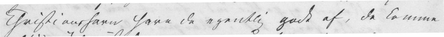Christianshavn have de egentlig godt af, de komme
